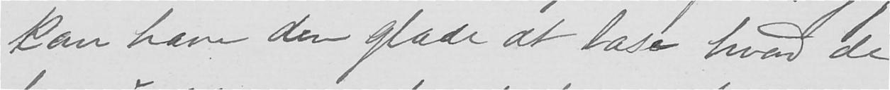kan have den glæde at læse hvad de
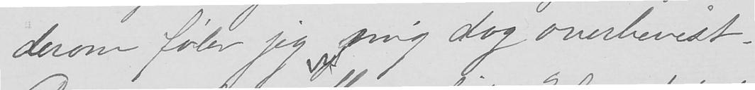derom føler jeg mig dog overbevist.
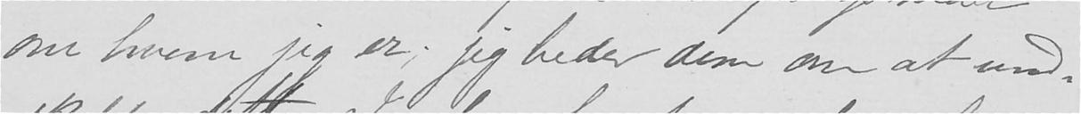om hvem jeg er; jeg beder dem om at und-
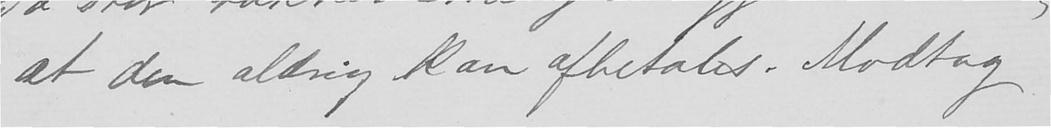at den aldrig kan afbetales. Modtag
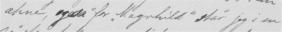ahne, også for "Magnhild" står jeg i en
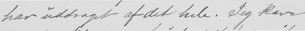hav uddraget af det hele. Jeg kan
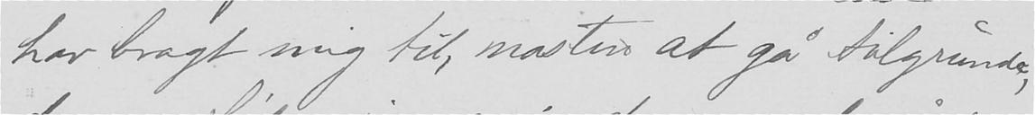har bragt mig til, næsten at gå tilgrunde,
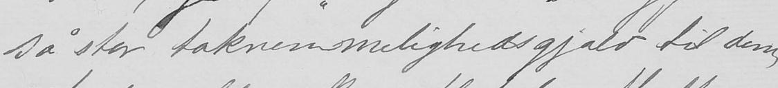så stor taknemmelighedsgjæld til dem,
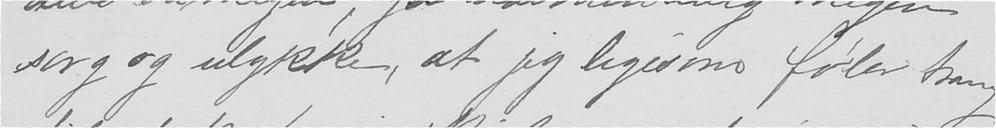sorg og ulykke, at jeg ligesom føler trang
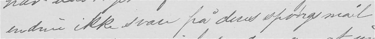endnu ikke svare på deres spørgsmål
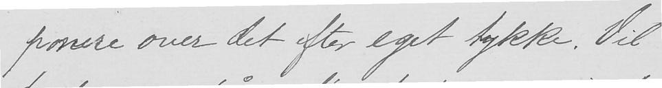ponere over det efter eget tykke. Vil
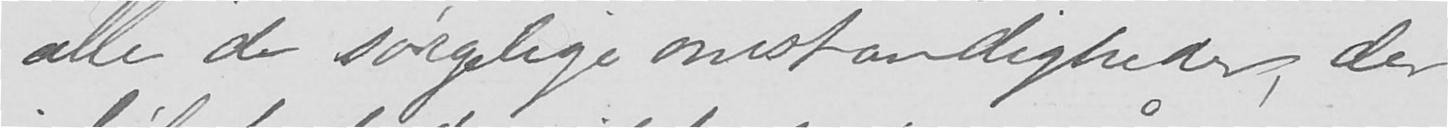alle de sørgelige omstændigheder, der
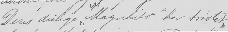Deres deilige "Magnhild" har trøstet,
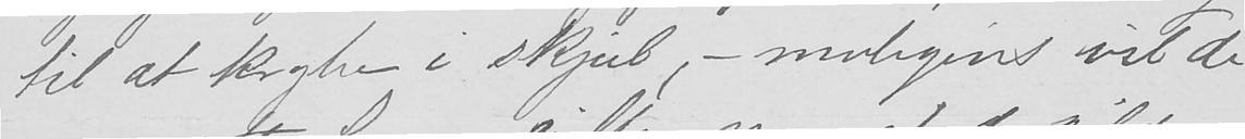til at krybe i skjul, - muligens vil de
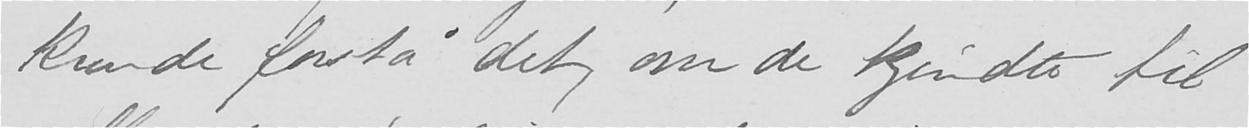kunde forstå det, om de kjendte til
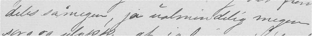deles så megen, ja ualmindelig megen
